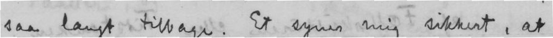saa langt tilbage. Et synes mig sikkert, at
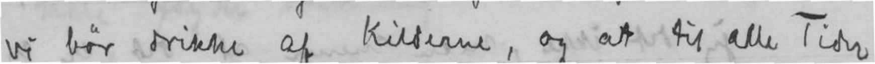vi bør drikke af Kilderne, og at til alle Tider
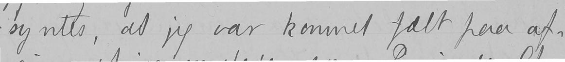syntes, at jeg var kommet fælt paa af-
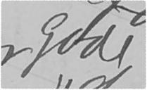gode
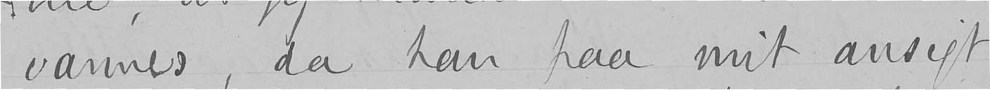vannes, da han paa mit ansigt
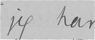jeg har
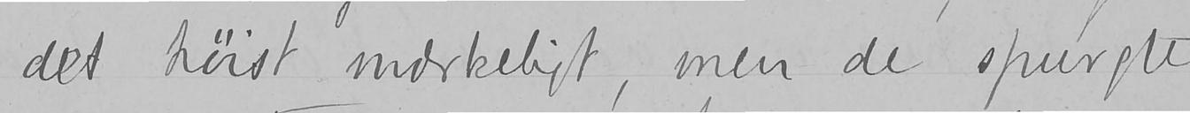det høist mærkeligt, men de spurgte
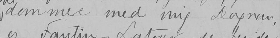dommere med mig Dagnan,
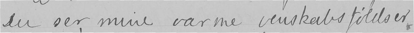Du ser, mine varme venskabsfølelser
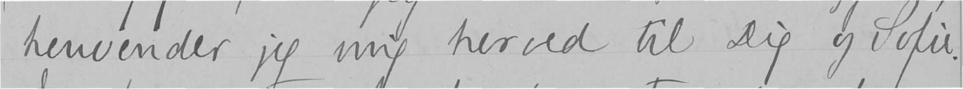henvender jeg mig herved til Dig og Sofie.
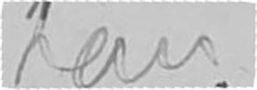han
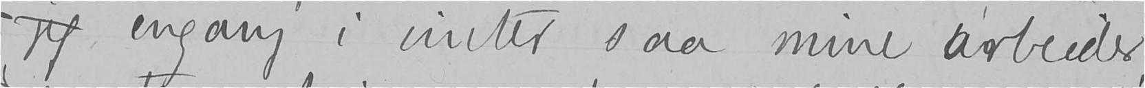jeg engang i vinter saa mine arbeider,
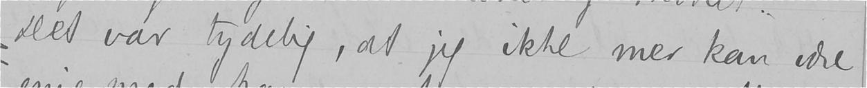Det var tydelig, at jeg ikke mer kan være
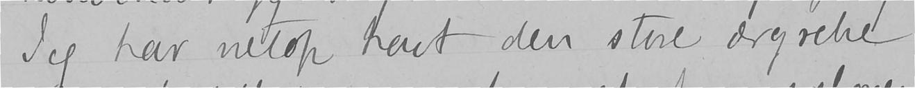Jeg har netop havt den store ærgrelse
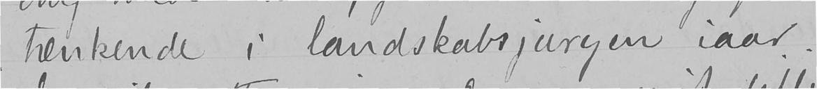tænkende i landskabsjuryen iaar.
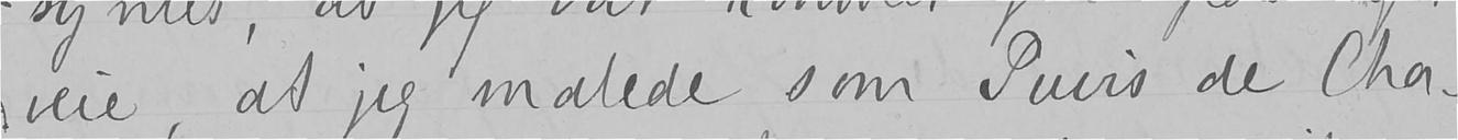veie, at jeg malede som Puvis de Cha-
